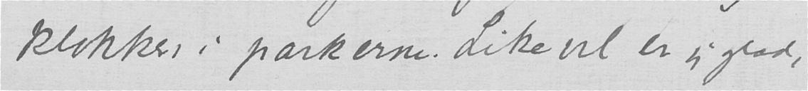klokker i parkerne. Likevel er jeg glad,
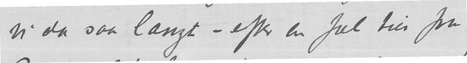vi da saa langt – efter en fæl tur fra
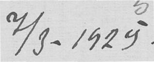7/3-1925
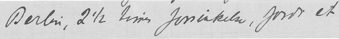Berlin, 2½ times forsinkelse, fordi et
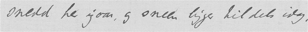snedd her igaar, og sneen ligger tildels idag,
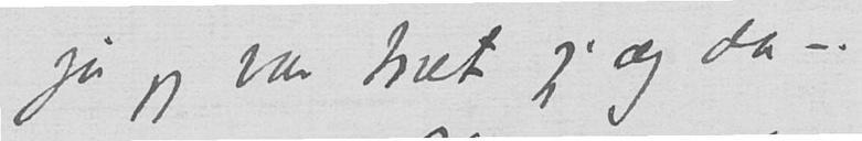ja jeg var træt jeg og da –.
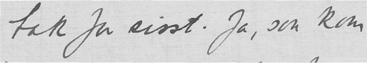tak for sisst. Ja, saa kom
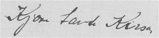Kjære tante Kirsa,
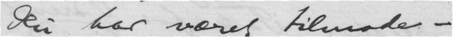Du har været tilmode -
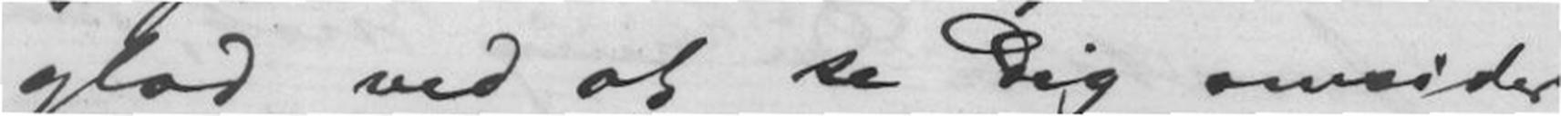glad ved at se Dig omsider
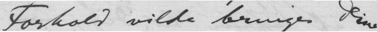Forhold vilde bringe Dine
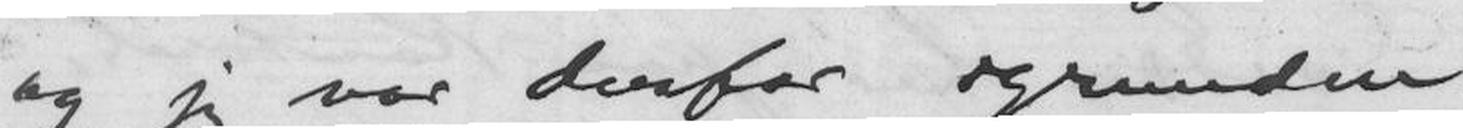og jeg var derfor igrunden
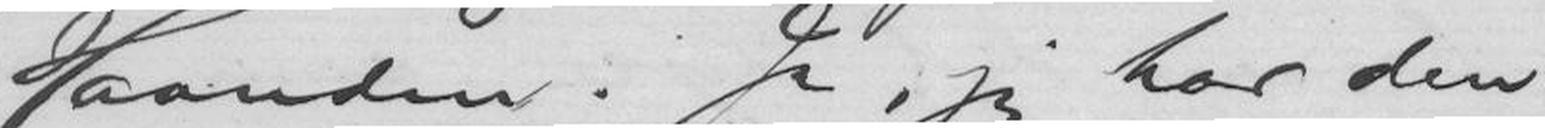Haanden. Ja, jeg har den
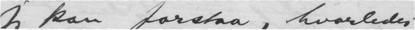jeg kan forstaa, hvorledes
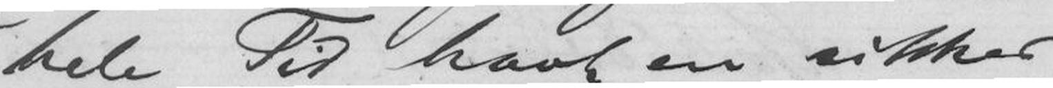hele Tid havt en sikker
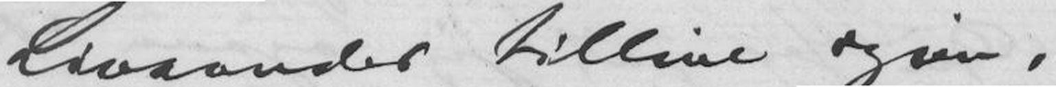Livaander tillive igjen,
